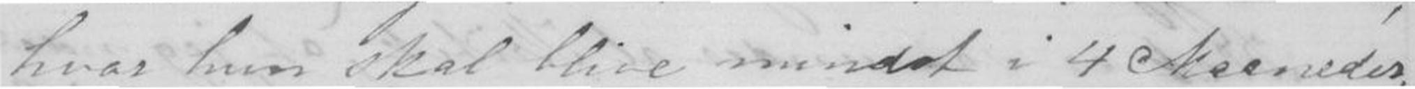hvor hun skal blive mindst i 4 Maaneder;
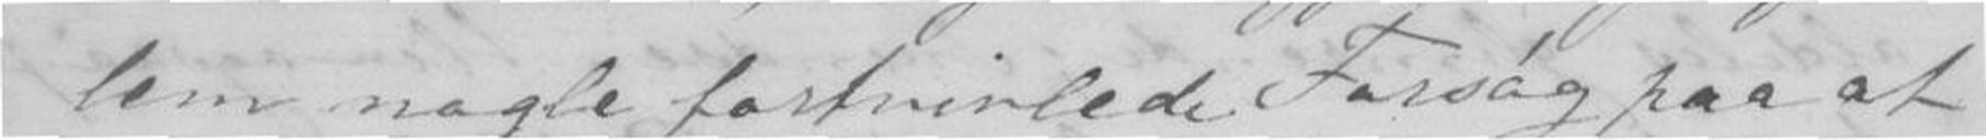lem nogle forvivlede Forsøg paa at
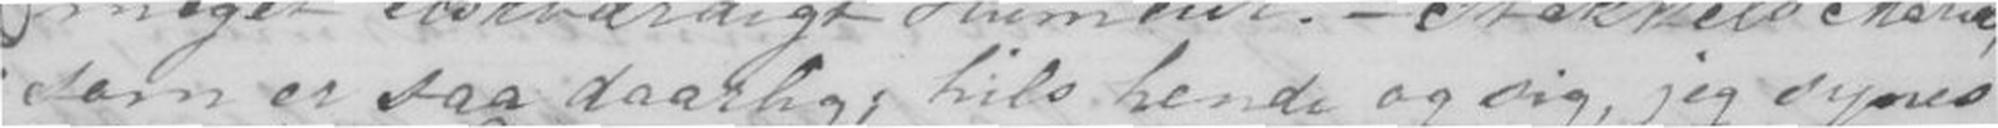som er saa daarlig, hils hende og sig, jeg synes
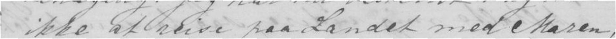ikke at reise paa lLandet med Maren
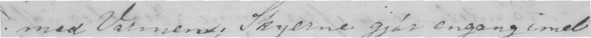med varmen; Skyerne gjør engang imel-
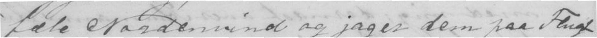fæle Nordenvind og jeger dem paa Flugt
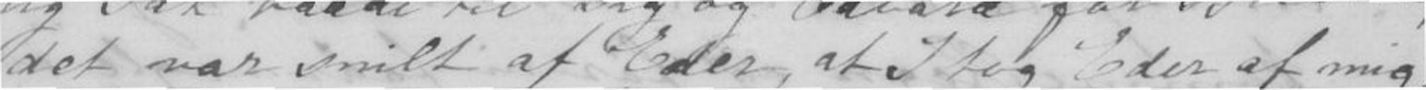det var snilt af Eder, at I tog Eder af mig,
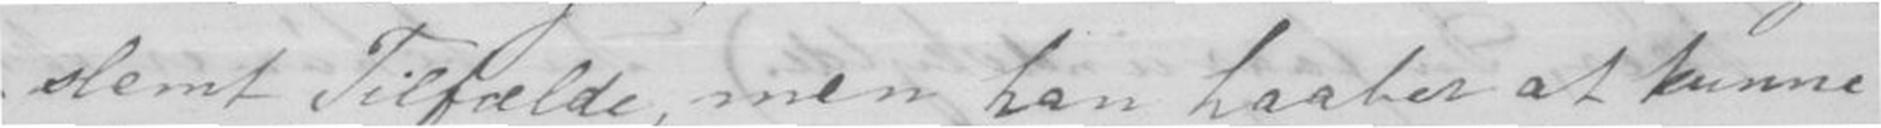slemt Tilfælde, men han haaber at kunne
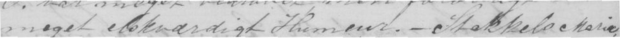meget elskværdigt Humuer. - Stakkels Marie,
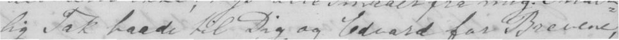lig Tak baade til Dig og Edvard for Brevene,
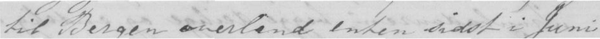til Bergen overland enten sidst i Juni
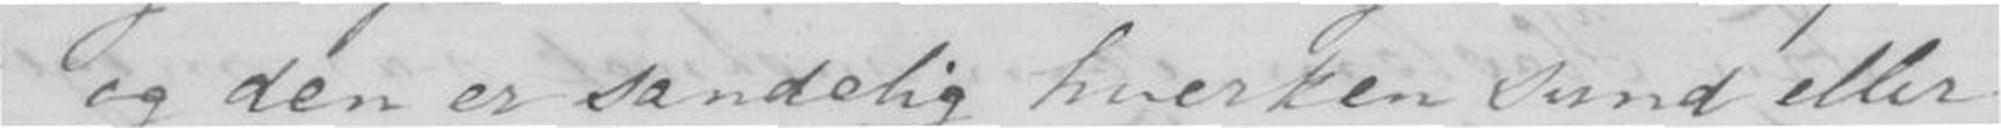og den er sandelig hverken sund eller
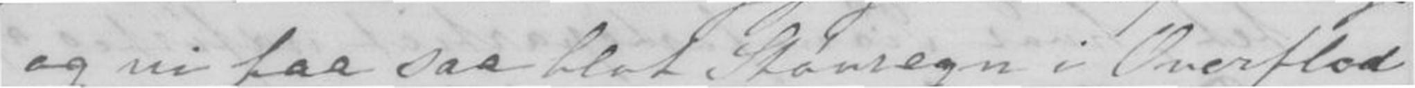og vi faa saa blot Støvregn i Overflod
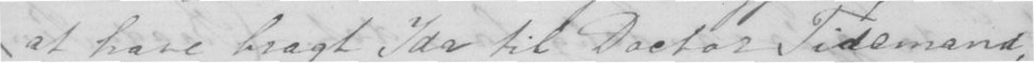at have Ida til Doctor Tidemand,
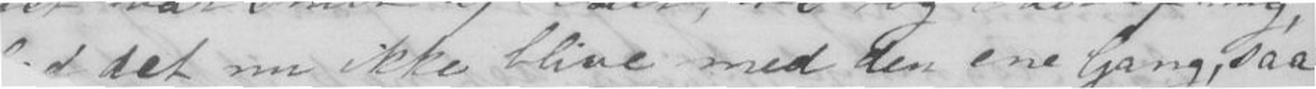det nu ikke blive med den ene Gang, saa
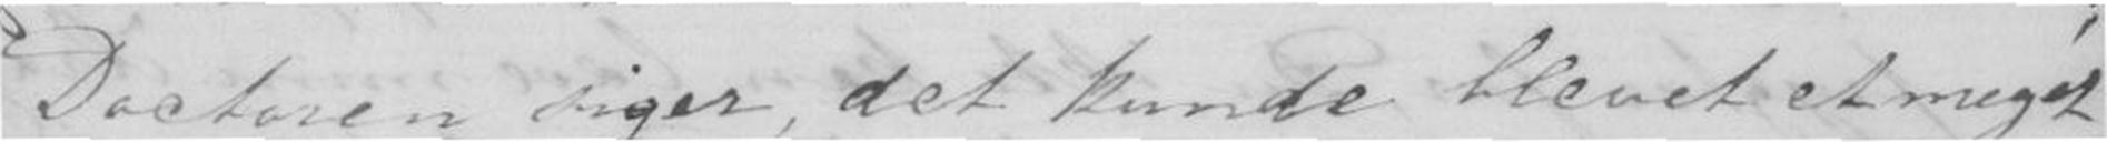Doctoren siger, det kunde blevet et meget
